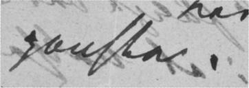ganske.
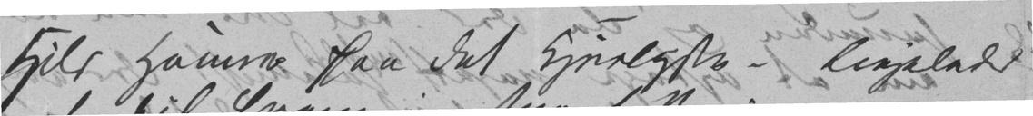Hils Hanne paa det Kjerligste – ligeledes
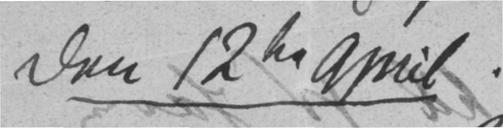Den 12te April.
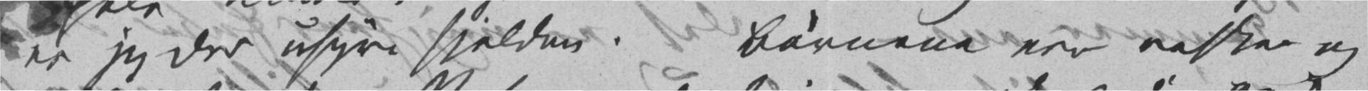er jeg der uhyre sjelden. Børnene ere raske og
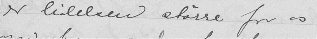er lidelsen større for os
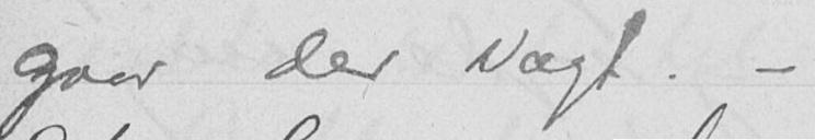gaar der vagt. -
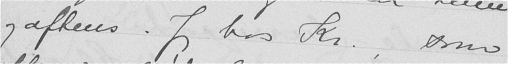og aftens. Jeg hos Kr. som
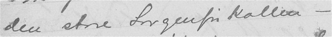den store Sorgenfrikollen -
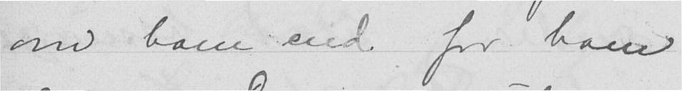om ham end for ham
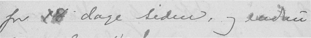for 14 dage siden, og endnu
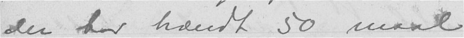der har brændt 50 maal
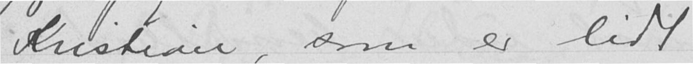Kristian, som er lidt
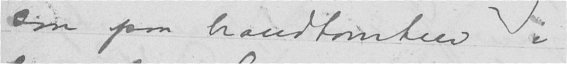saa paa brandtomten i
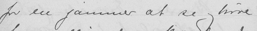for en jammer at se og høre
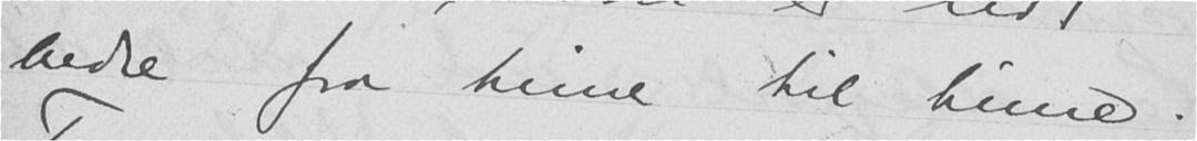bedre fra time til time.
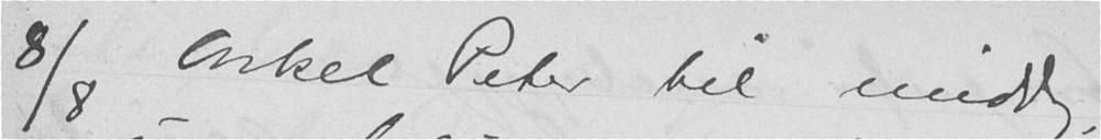8/8 Onkel Peter til middag,
